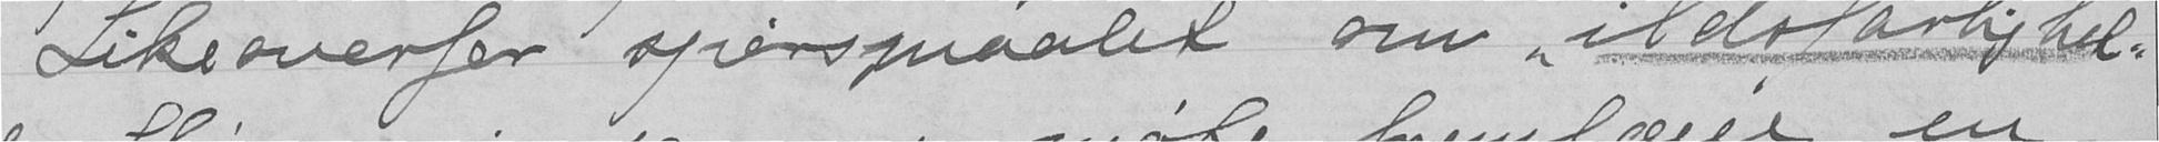Likeoverfor spørsmaalet om "ildsfavlighet"
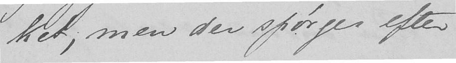ket, men der spørges efter
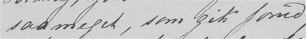saameget, som gik forud
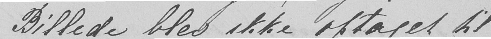Billede blev ikke optaget til
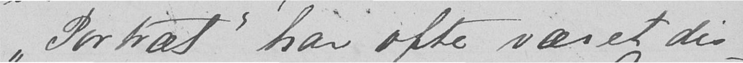"Portræt" har ofte været dis-
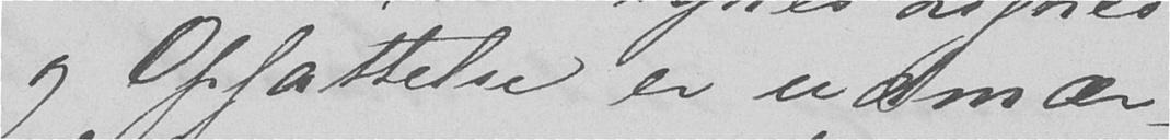og Opfattelse er udmær-
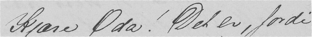Kjære Oda! Det er, fordi
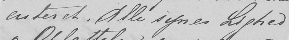cuteret. Alle synes Lighed
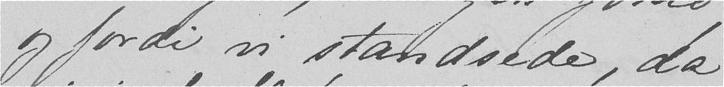og fordi vi standsede, da
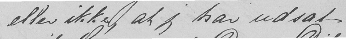eller ikke, at jeg har udsat
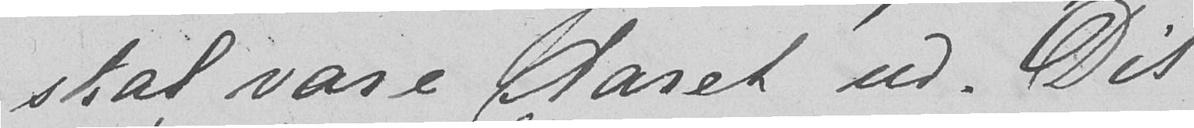skal vare Aaret ud. Dit
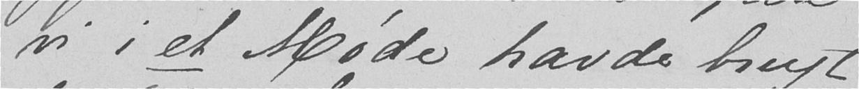vi i et Møde havde brugt
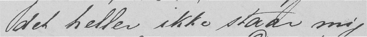det heller ikke staar mig
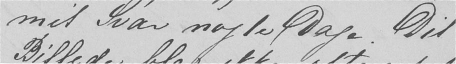mit Svar nogle Dage. Dit
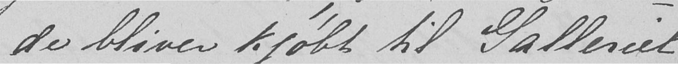de bliver kjøbt til Galleriet
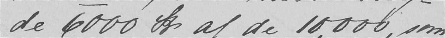de 6000 Kr af de 10,000, som
